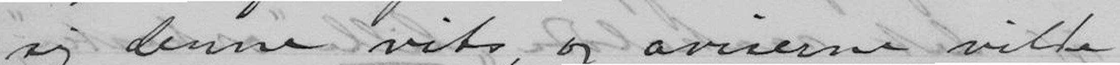sig denne vits, og aviserne vilde
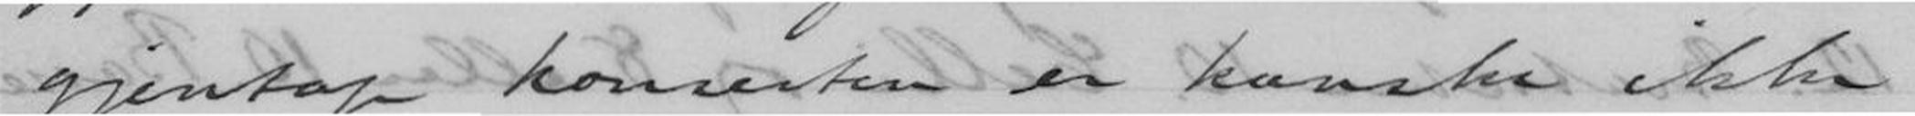gjentage konserten er kanske ikke
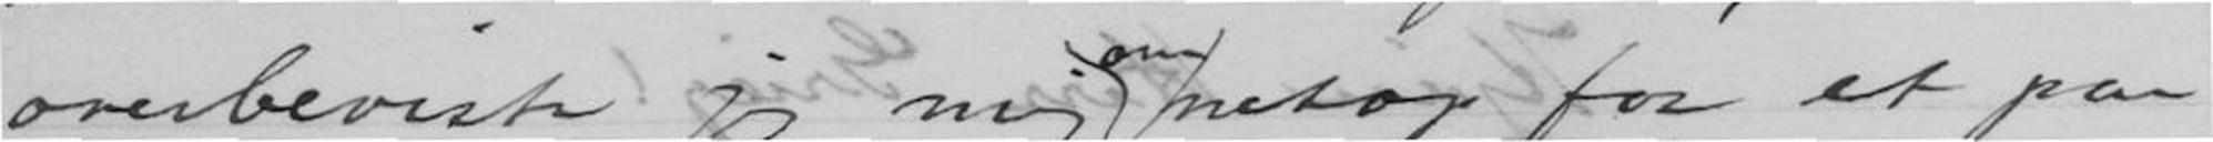overbeviste jeg mig netop for et par
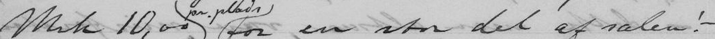Mrk 10,00 for en stor del af salen! -
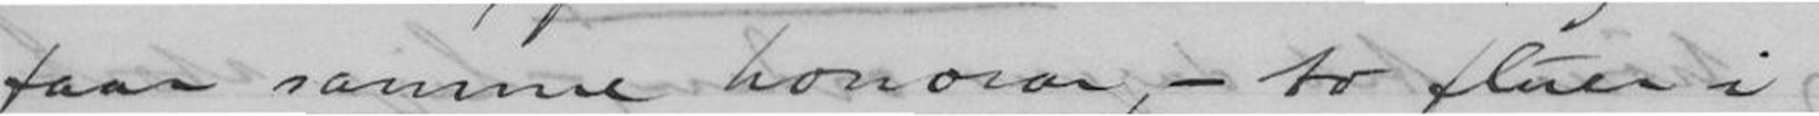faar samme honorar, - to fluer i
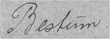Bestum.
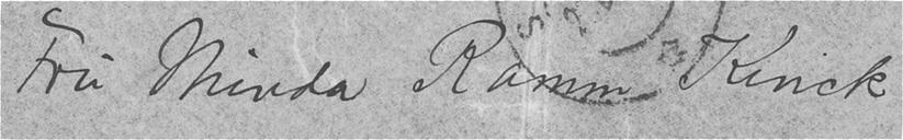Fru Minda Ramm Kinck
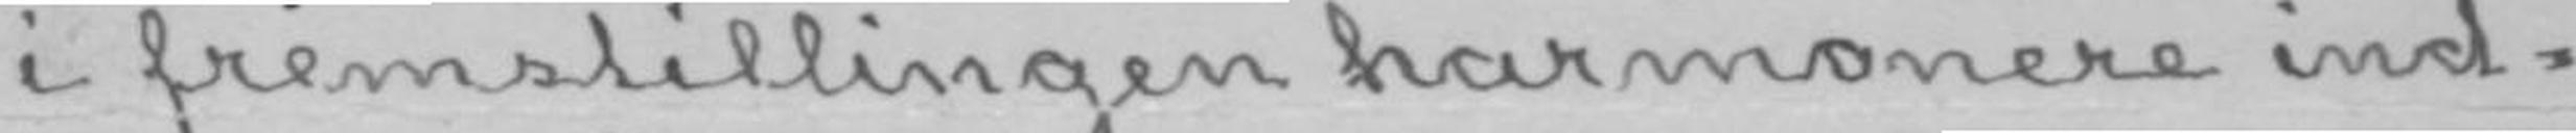i fremstillingen harmonere ind-
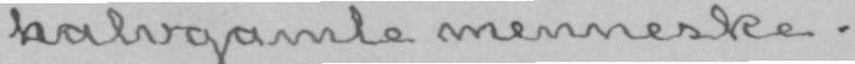halvgamle menneske.
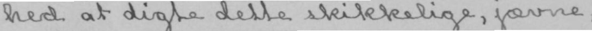hed at digte dette skikkelige, jævne,
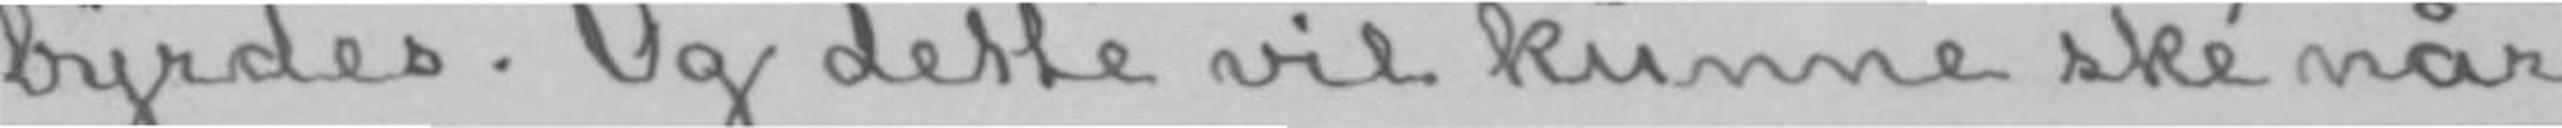byrdes. Og dette vil kunne ské når
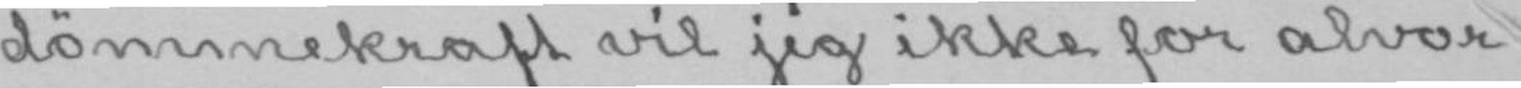dømmekraft vil jeg ikke for alvor
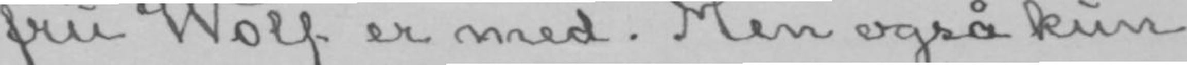fru Wolf er med. Men også kun
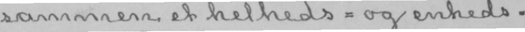sammen et helheds- og enheds-
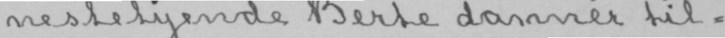nestetyende Berte danner til-
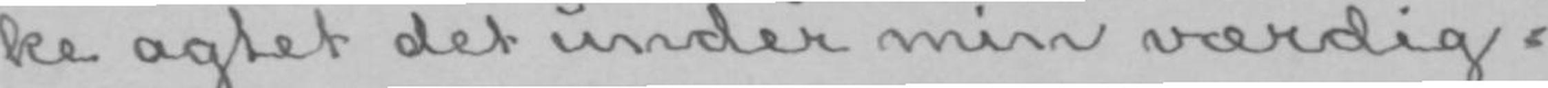ke agtet det under min værdig-
